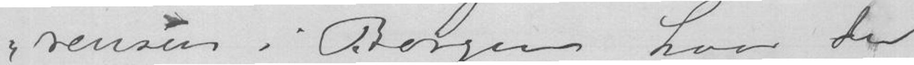ransen i Bergen hvor Du
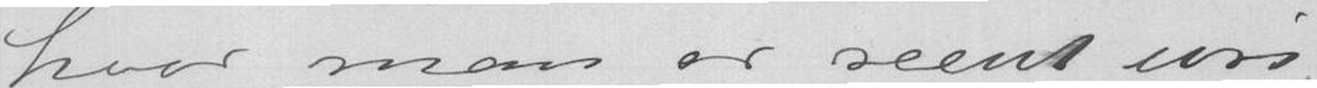hvor man er reent uri
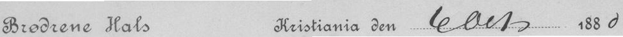Brødrene Hals Kristiania den 6 Oct 1880
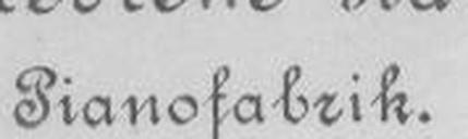Pianofabrik.
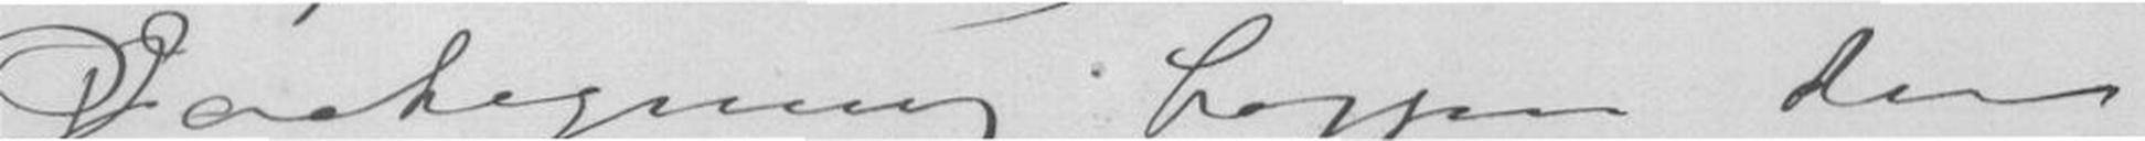Paategning bagpaa din
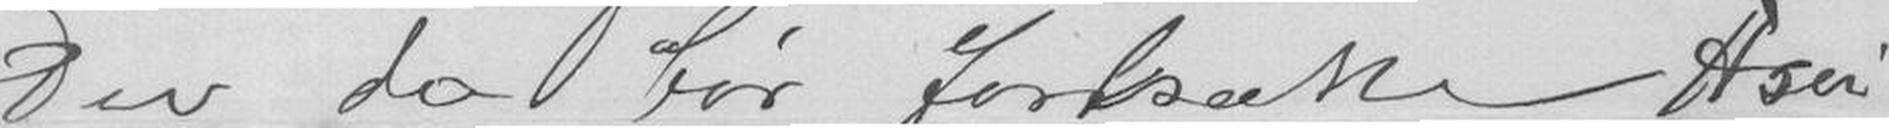Du da bør fortsætte Asu-
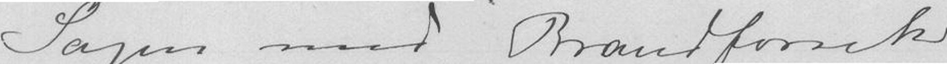Sagen med Brandforsik-
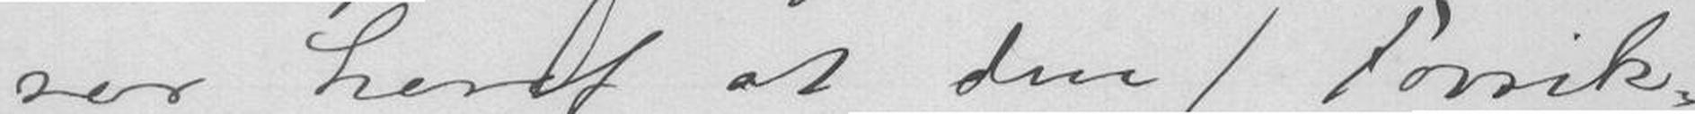ser heraf at den (Forsik-
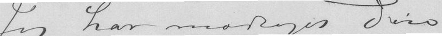Jeg har modtaget Din
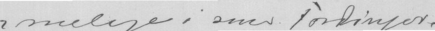melige i sine Fordringer.
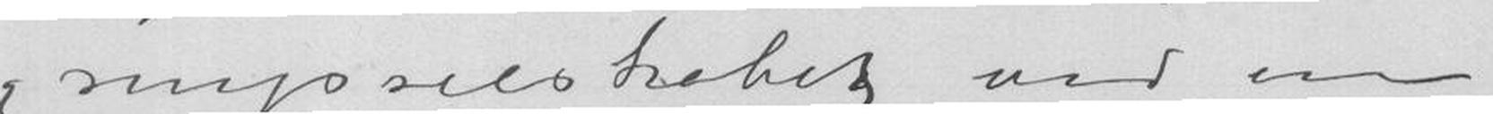ringsselskabet ved en
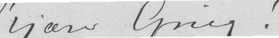Kjære Grieg!
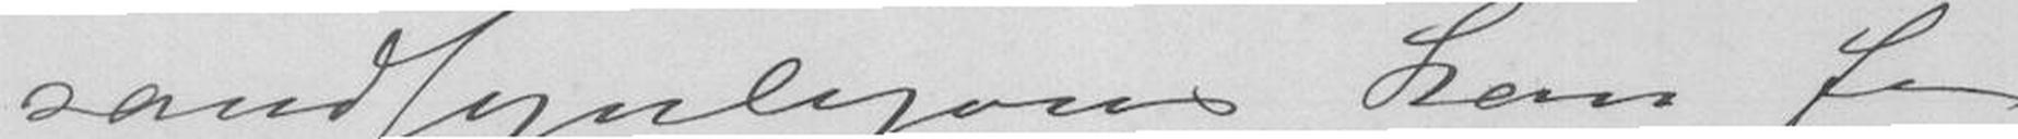sandsynligvis kan faa
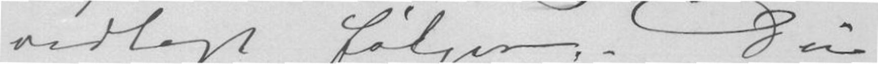vedlagt følger. Du
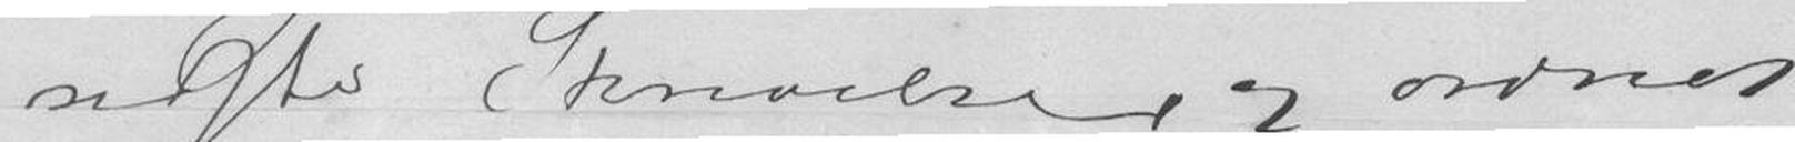sidste Skrivelse og ordnet
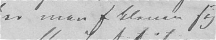er man bleven sig
